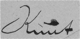Knut
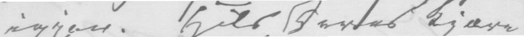igjen. Hils Deres kjære
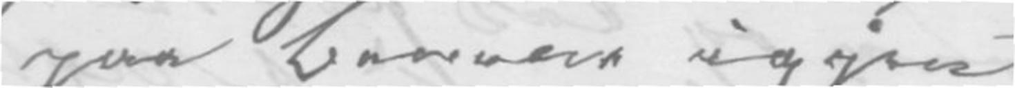paa benene igjen.
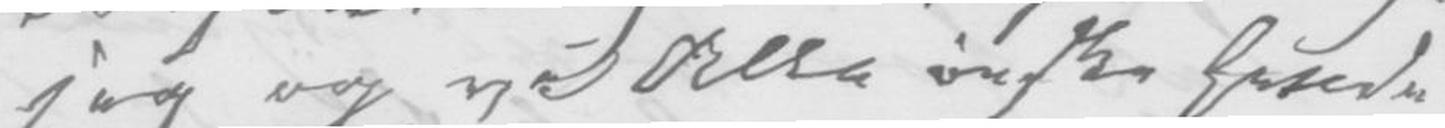jeg og vi Alle ønske hende
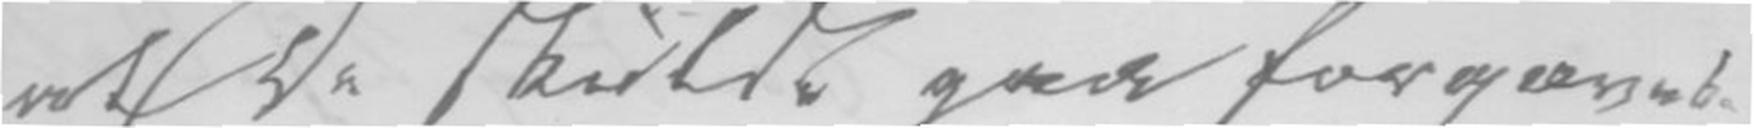at De skulde gaa forgæves.
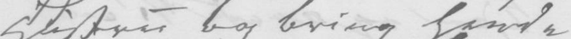Hustru og bring hende
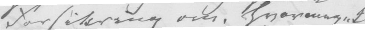Forsikring om, hvormeget
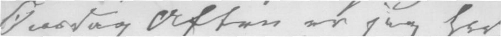Onsdag Aften er jeg her
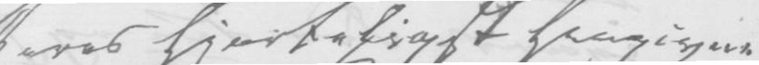Deres hjertligst hengivne
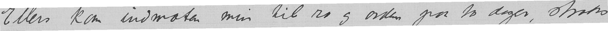Ellers kom indmaten min til ro og orden paa to dager, straks
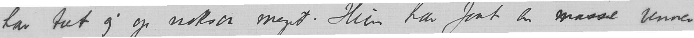har tat sig op noksaa meget. Hun har faat en masse venner
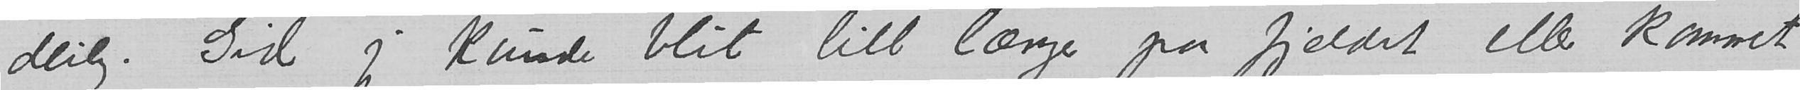deilig. Gid jeg kunde blit litt længe paa fjeldet eller kommet
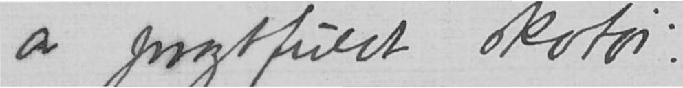av pragtfuldt skotøi:
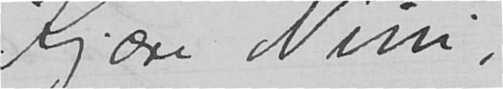Kjære Nini
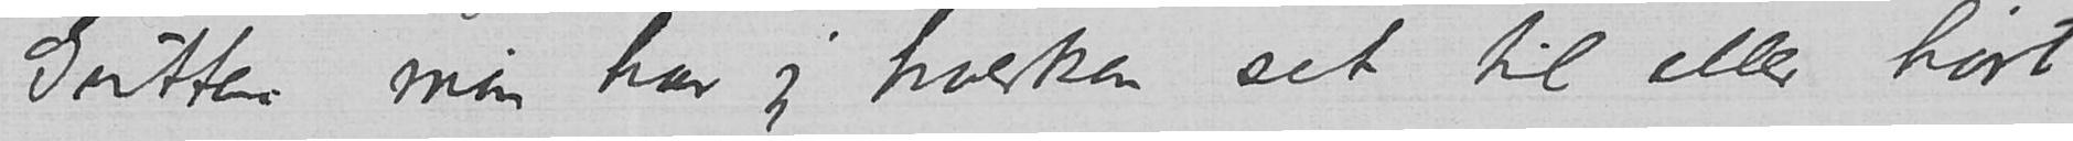Gutten min har jeg hverken set til eller hørt
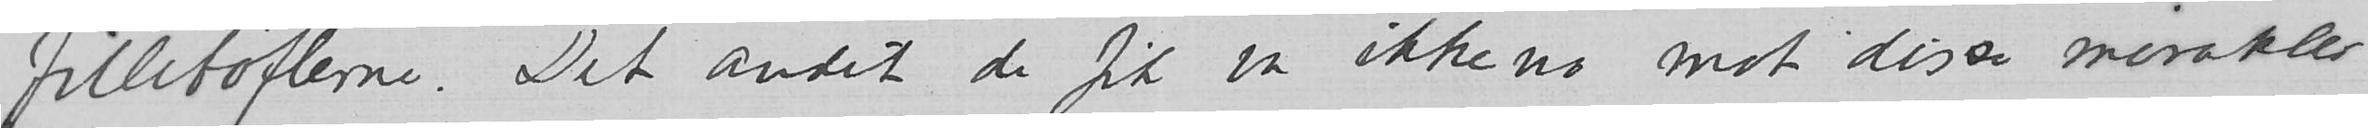filletøflerne. Det andet de fik var ikke no mot disse mirakler
